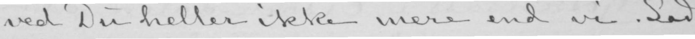ved Du heller ikke mere end vi. Lad
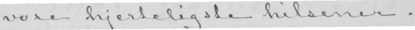vore hjerteligste hilsener.
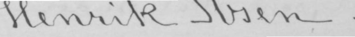Henrik Ibsen.
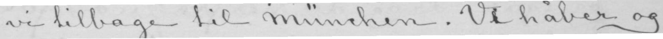vi tilbage til München. Vi håber og
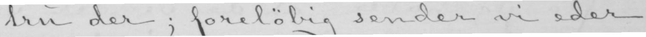tru der; foreløbig sender vi eder
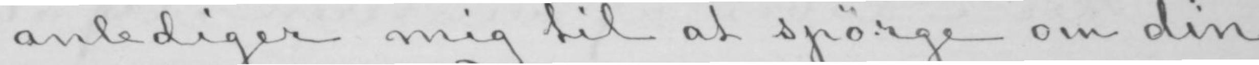anlediger mig til at spørge om din
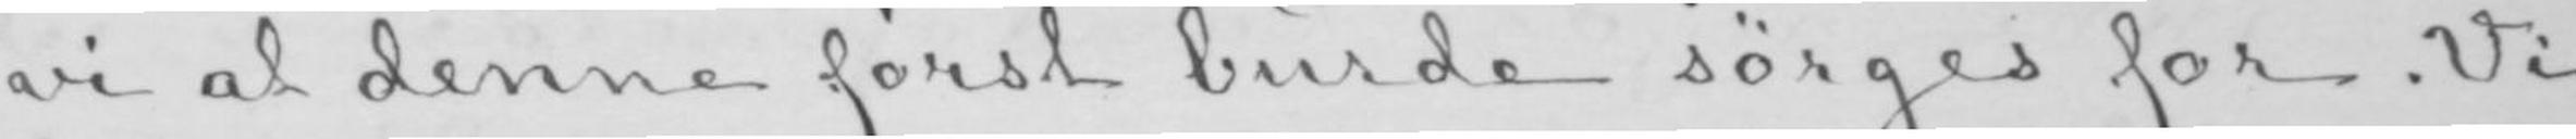vi at denne først burde sørges for. Vi
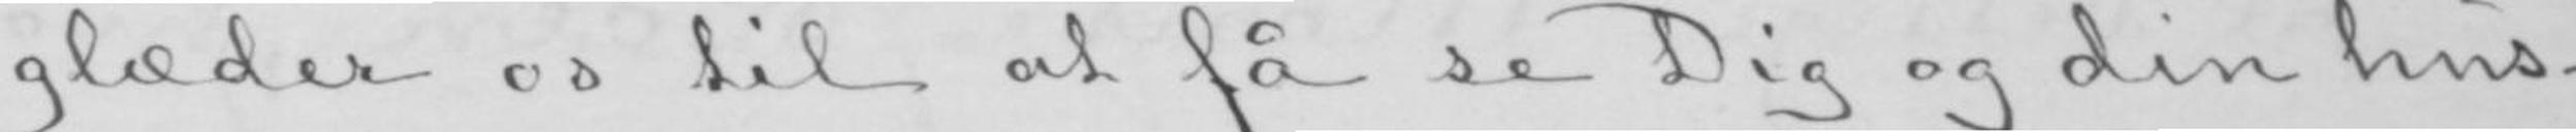glæder os til at få se Dig og din hus-
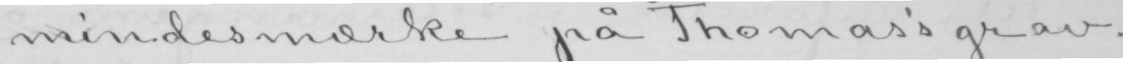mindesmærke på Thomas's grav.
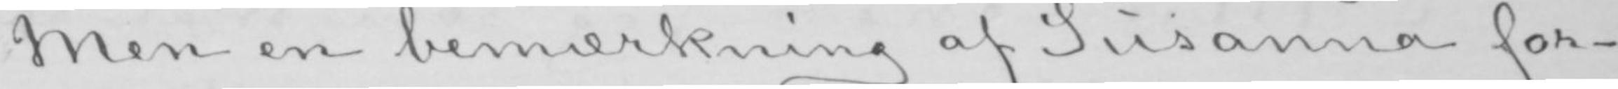Men en bemærkning af Susanna for-
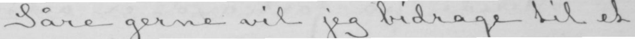Såre gerne vil jeg bidrage til et
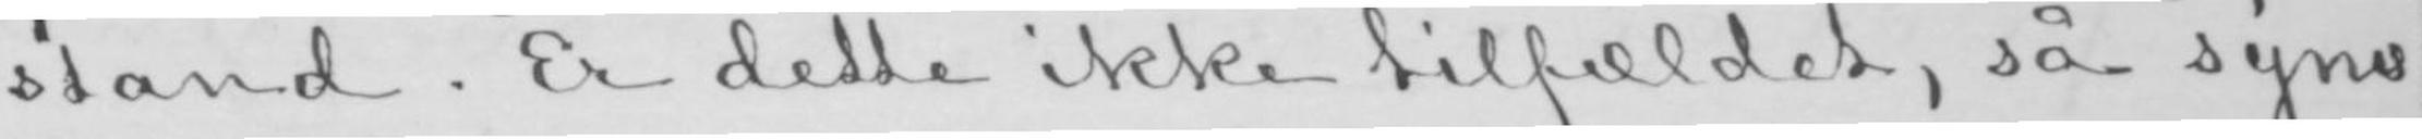stand. Er dette ikke tilfældet, så synes
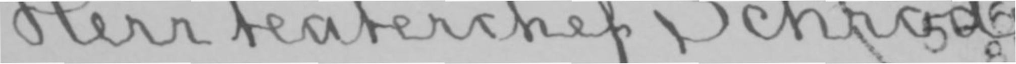Herr teaterchef Schrøder.
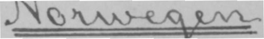Norwegen.
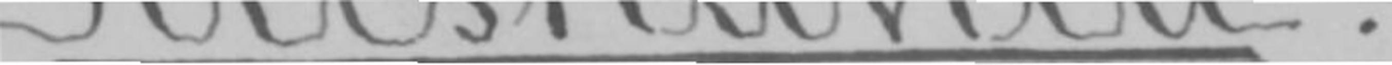Kristiania.
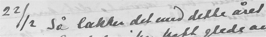22/12 Så lukker det med dette året
